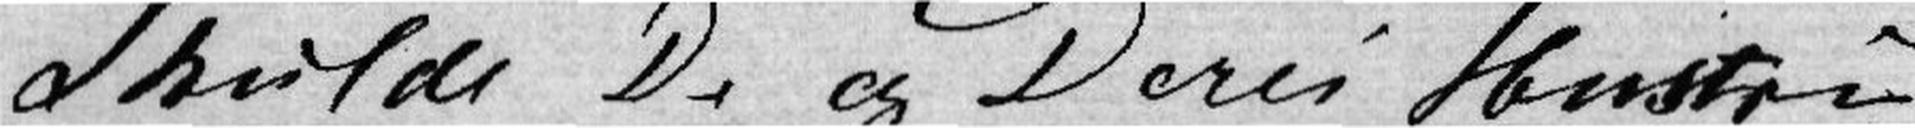Skulde De og Deres Hustru
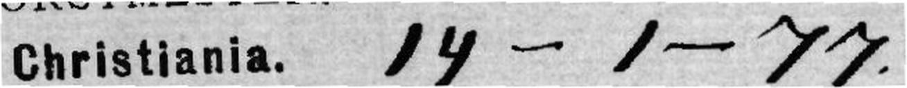Christiania 14-1-77.
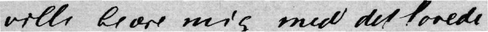ville beære mig med det lovede
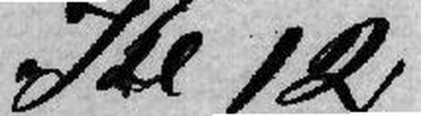Kl 12
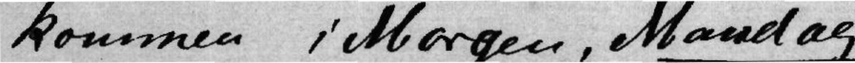kommen i Morgen, Mandag
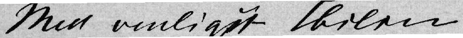Med venligst Hilsen
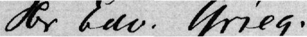Hr. Edv. Grieg.
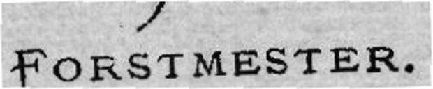FORSTMESTER
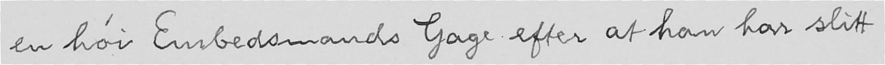en høi Embedsmands Gage efter at han har slitt
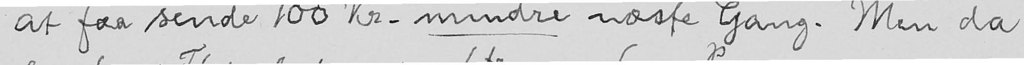at faa sende 100 Kr. mindre næste Gang. Men da
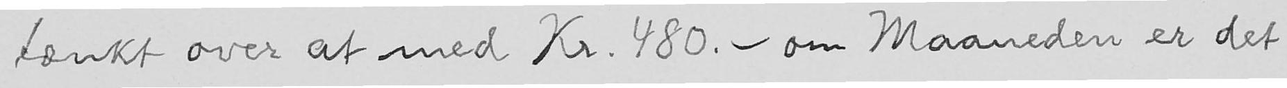tænkt over at med Kr. 480. - om Maaneden er det
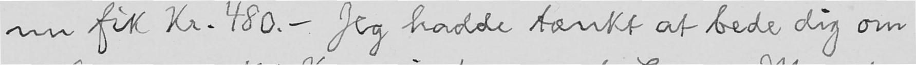nu fik Kr. 480. - Jeg hadde tænkt at bede dig om
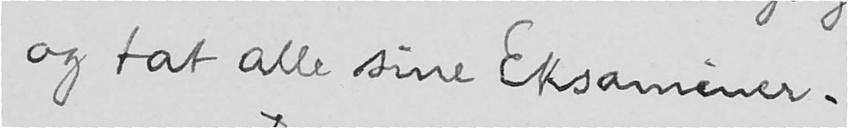og tat alle sine Eksaminer.
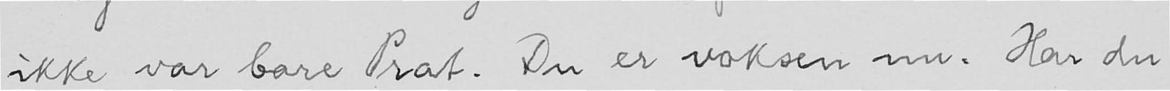ikke var bare Prat. Du er voksen nu. Har du
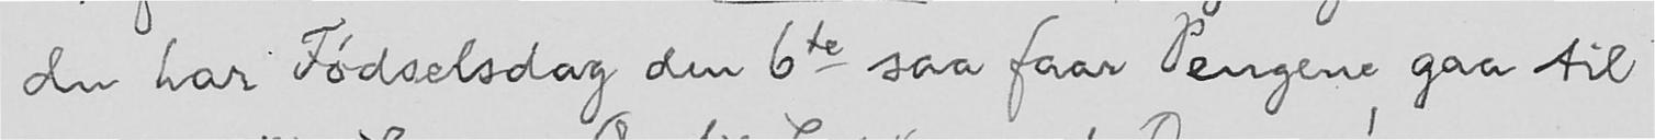du har Fødselsdag den 6te saa faar Pengene gaa til
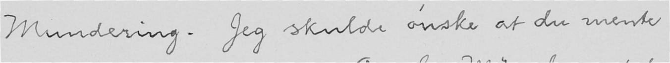Mundering. Jeg skulde ønske at du mente
